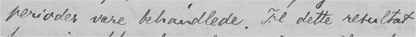perioder vare behandlede. Til dette resultat
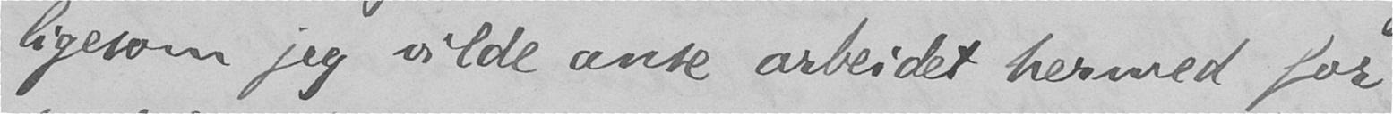ligesom jeg vilde anse arbeidet hermed for
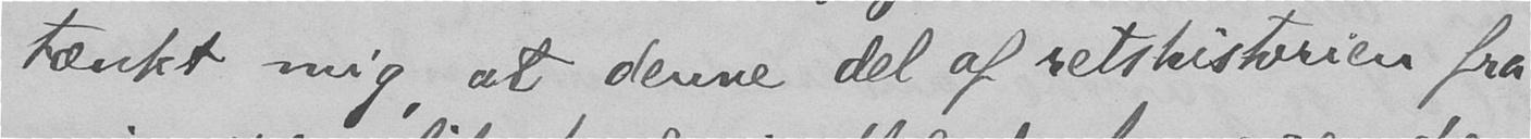tænkt mig, at denne del af retshistorien fra
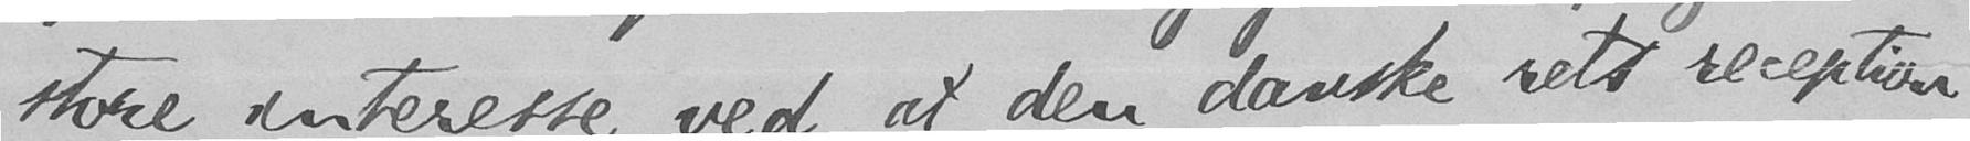store interesse ved, at den danske rets reception
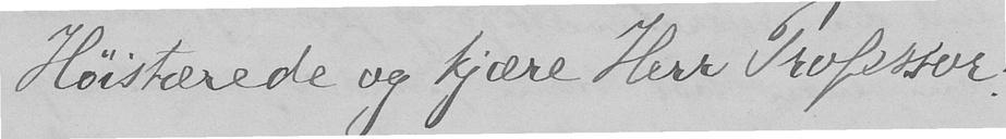Høistærede og kjære Herr Professor!
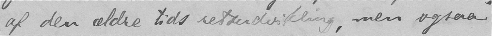af den ældre tids retsudvikling, men ogsaa
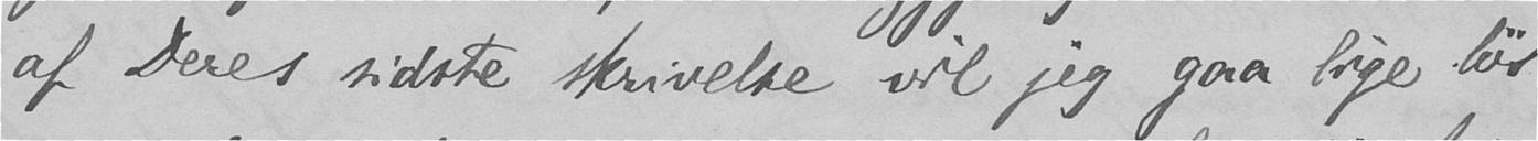af Deres sidste skrivelse vil jeg gaa lige løs
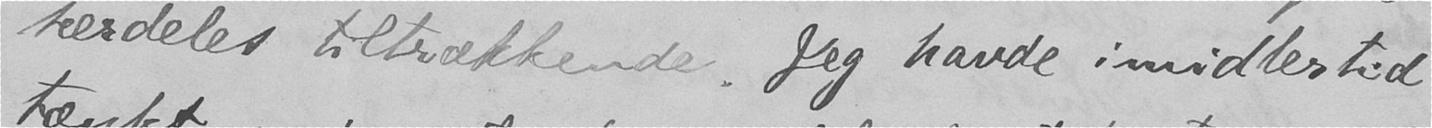særdeles tiltrækkende. Jeg havde imidlertid
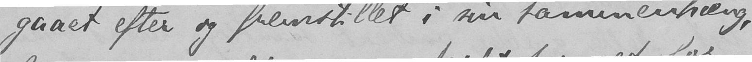gaaet efter og fremstillet i sin sammenhæng,
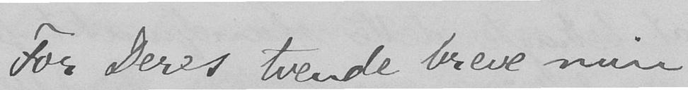For Deres tvende breve min
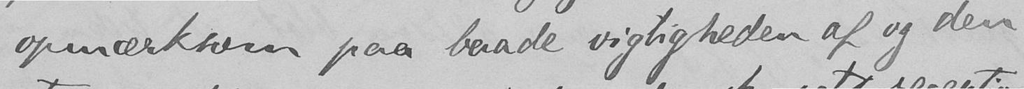opmærksom paa baade vigtigheden af og den
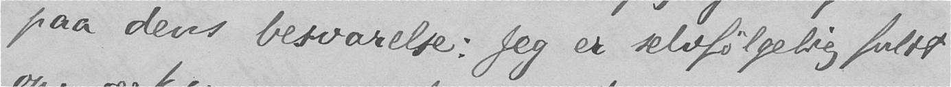paa dens besvarelse: Jeg er selvfølgelig fuldt
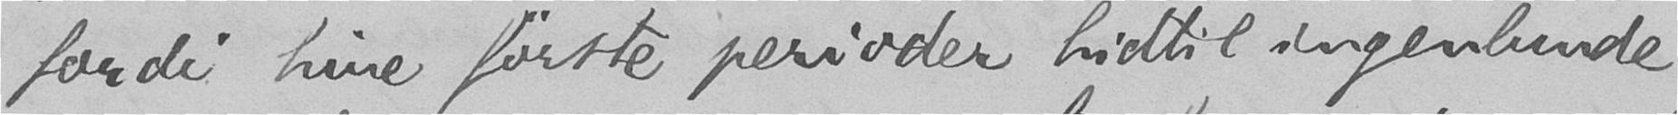fordi hine første perioder hidtil ingenlunde
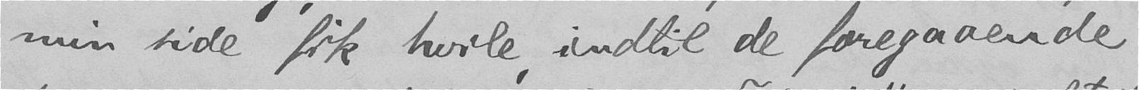min side fik hvile, indtil de foregaaende
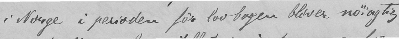i Norge i perioden før lovbogen bliver nøiagtig
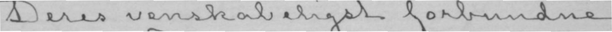Deres venskabeligst forbundne
